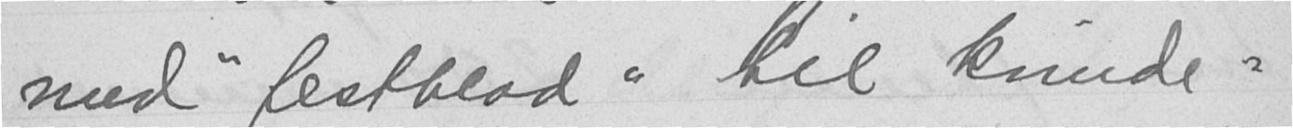med "festblod" til kvinde-
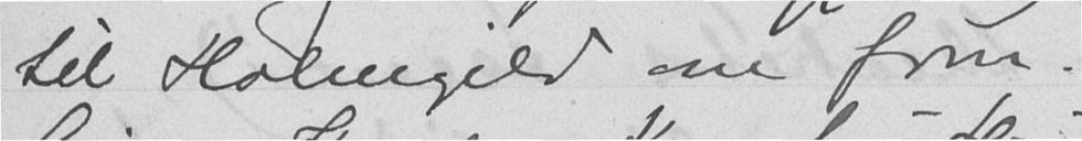til Holmgild om form.
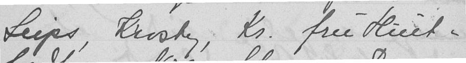Seips, Krosby, Kr. fru Huit-
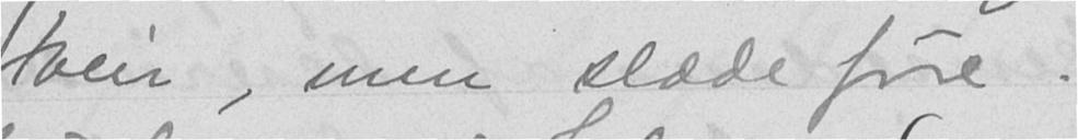veir, men slædeføre.
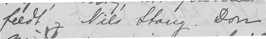feldt og Nils Stang. Don
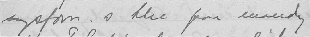sagsforn.s blie paa mandag
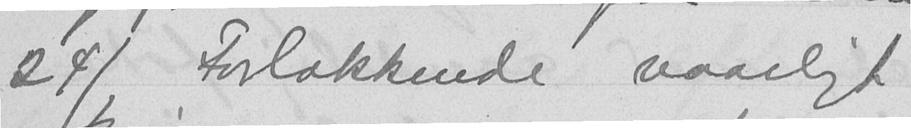24/1 Forlokkende vaarligt
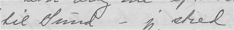til Sund - jeg stred
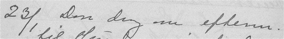23/1 Don drog om efterm.
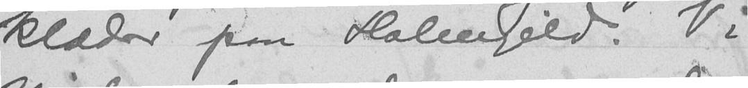klæder paa Holmgild. Vi
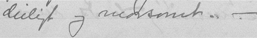deiligt og morsomt. -
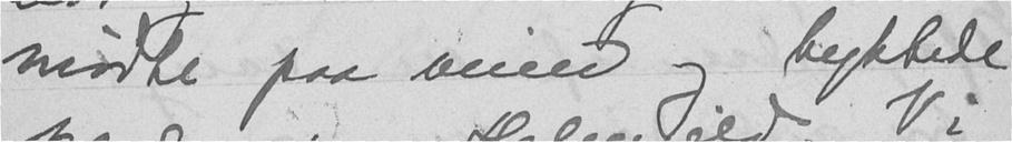mødte paa veien og byttede
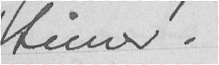timer.
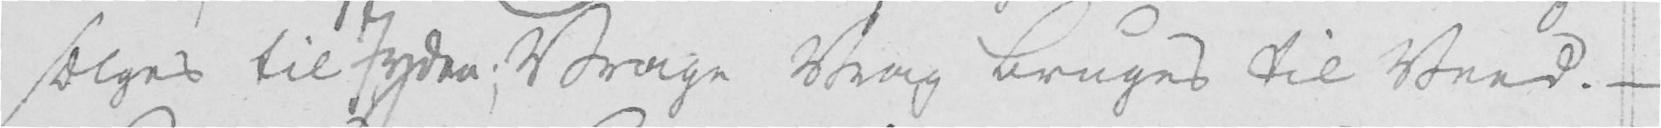sælges til Jyden; Vrage Vrag bruges til Veed. -
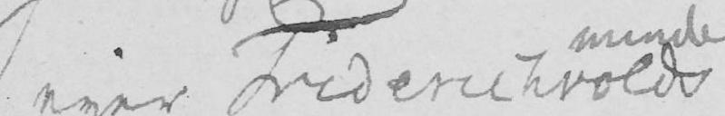eyer Friderichvolds
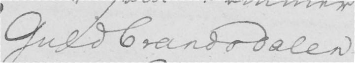Guldbrandsdalen
